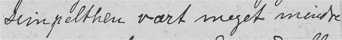simpelthen vært meget mindre
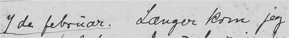7de februar. Længer kom jeg
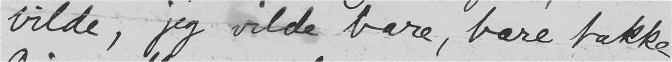vilde, jeg vilde bare, bare takke
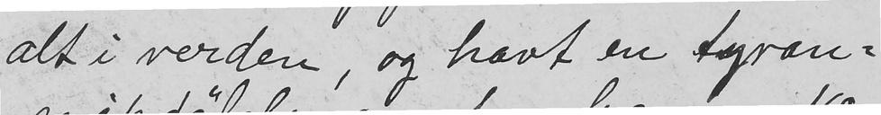alt i verden, og havt en tyran-
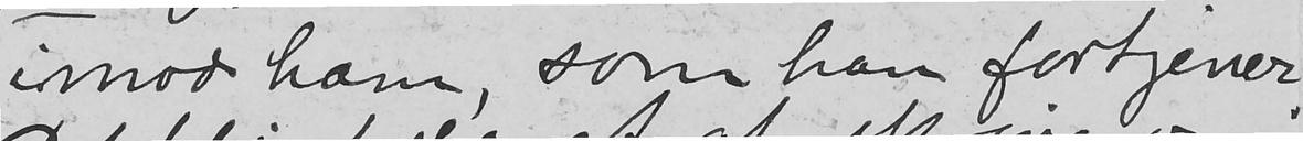imod ham, som han fortjener.
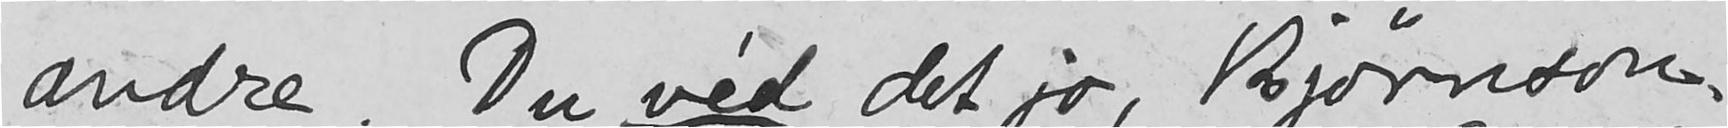andre. Du véd det jo, Bjørnson.
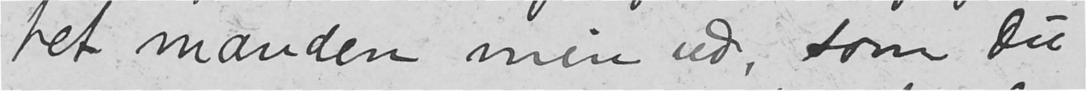tet manden min ud, som Du
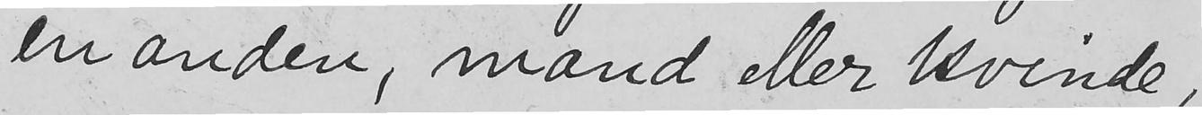en anden, mand eler kvinde,
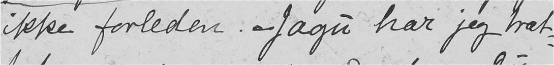ikke forleden. - Jagu har jeg træt
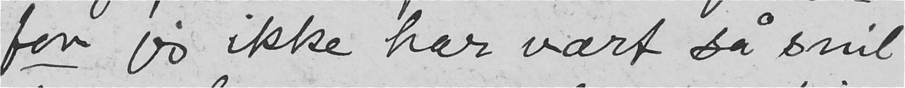for jeg ikke har vært så snil
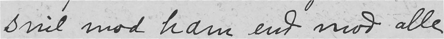snil mod ham end mod alle
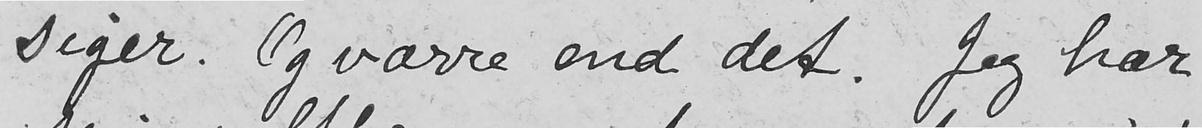siger. Og værre end det. Jeg har
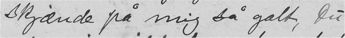skjænde på mig så galt, Du
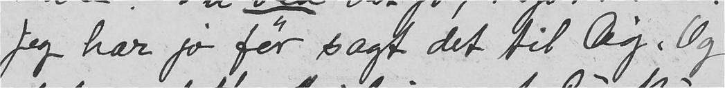Jeg har jo før sagt det til Dig. Og
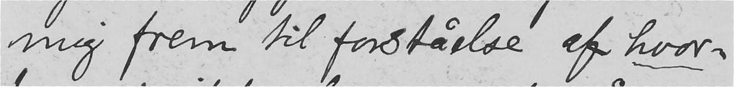mig frem til forståelse af hvor-
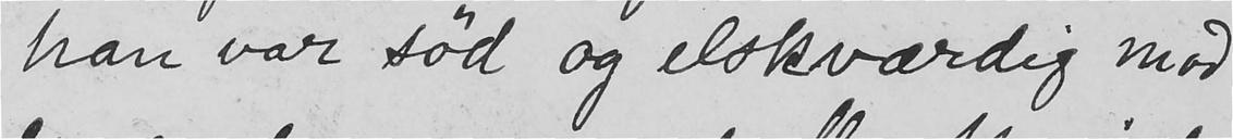han var sød og elskværdig mod
